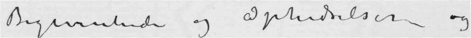Begivenheder og Ophidselser og
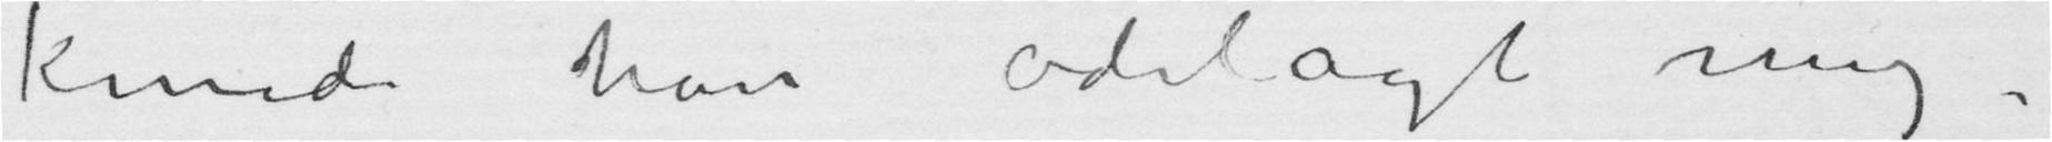kunde have ødelagt mig.
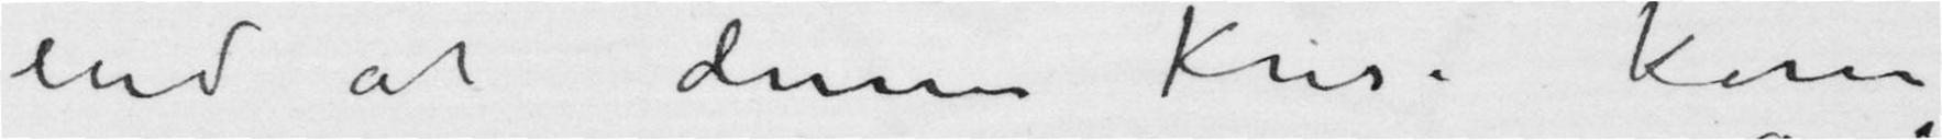end at denne Krise kom
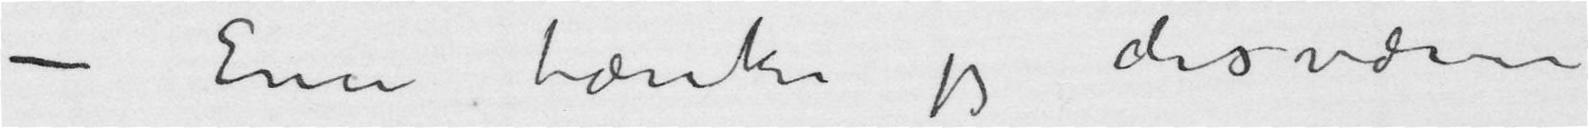– Ennu tænker jeg desværre
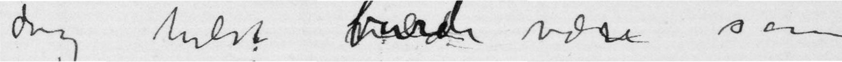dog helst burde være som
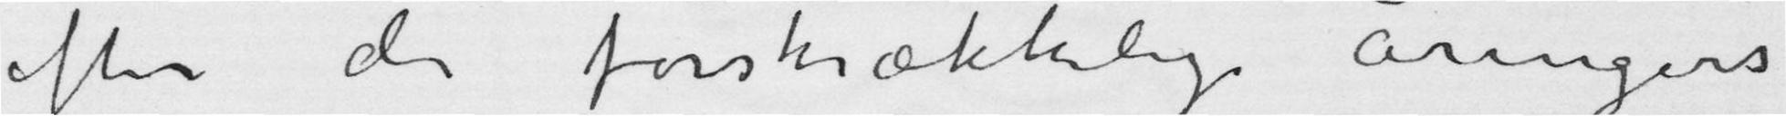efter de forskrækkelige åringers
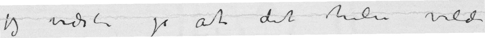jeg vidste jo at det hele vilde
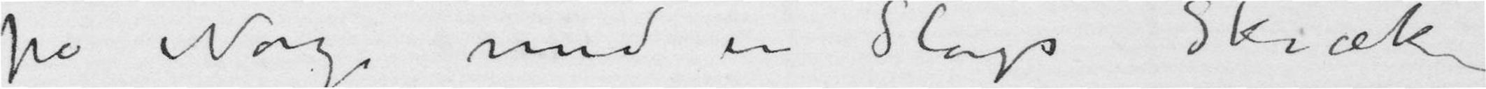på Norge med en Slags Skræk
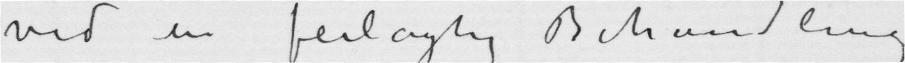ved en feilagtig Behandling
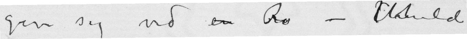give sig ved en Ro – Uheld
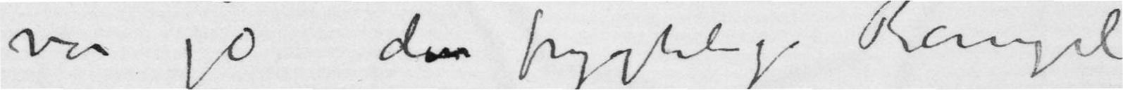var jo den frygtelige Rangel
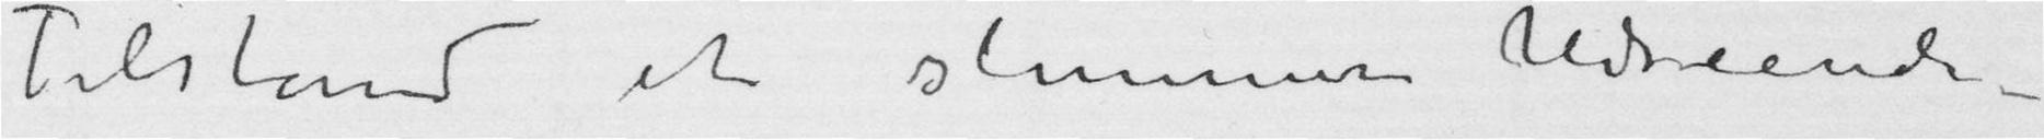Tilstand et slemmere Udseende –
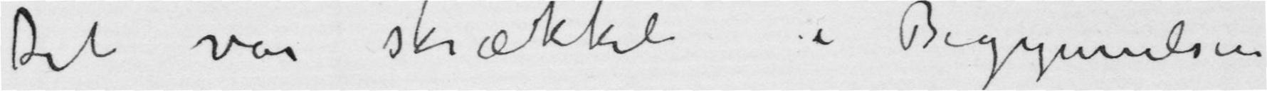Det var skrækkeli i Begynnelsen
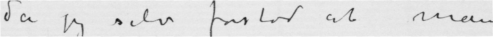da jeg selv forstod at man
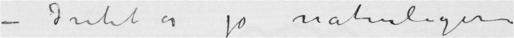– Intet er jo naturligere
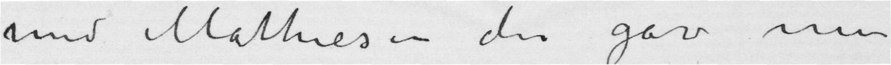med Mathiesen der gav min
